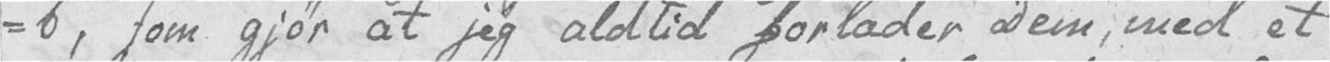-b, som gjør at jeg aldtid forlader Dem, med et
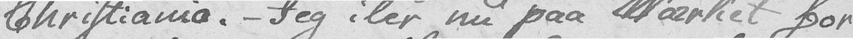Christiania. – Jeg iler nu paa Værket for
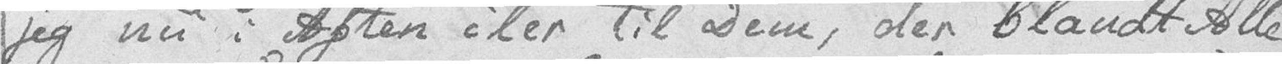jeg nu i Aften iler til Dem, der blandt Alle
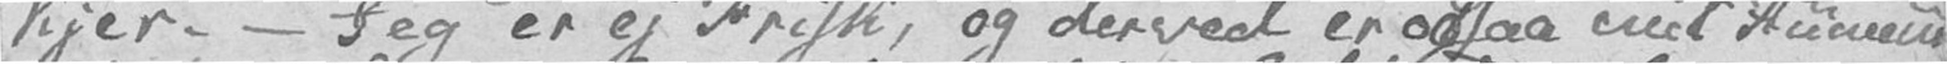kjer. – Jeg er ej Frisk, og derved er ogsaa mit Humeur
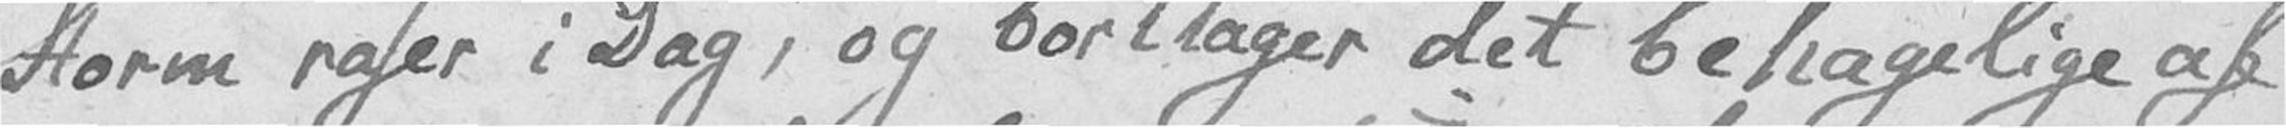Storm raser i Dag, og borttager det behagelige af
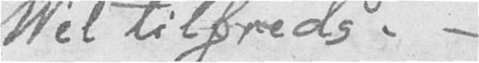Vel tilfreds. –
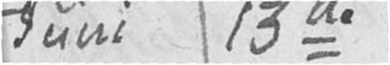Juni 13de
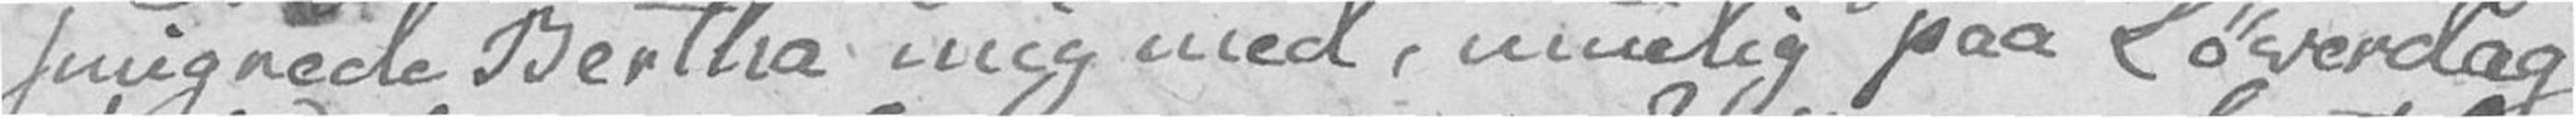smigrede Bertha mig med, muelig paa Løverdag
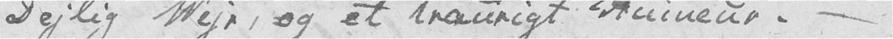Dejlig Vejr, og et traurigt Humeur. –
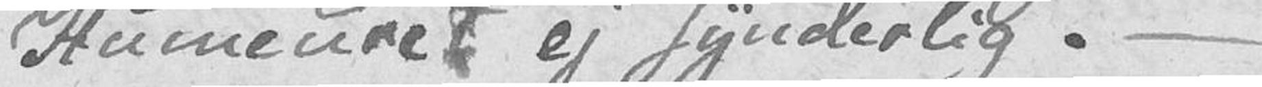Humeuret ej synderlig. –
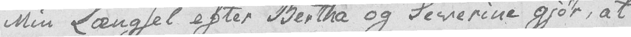Min Længsel efter Bertha og Severine gjør, at
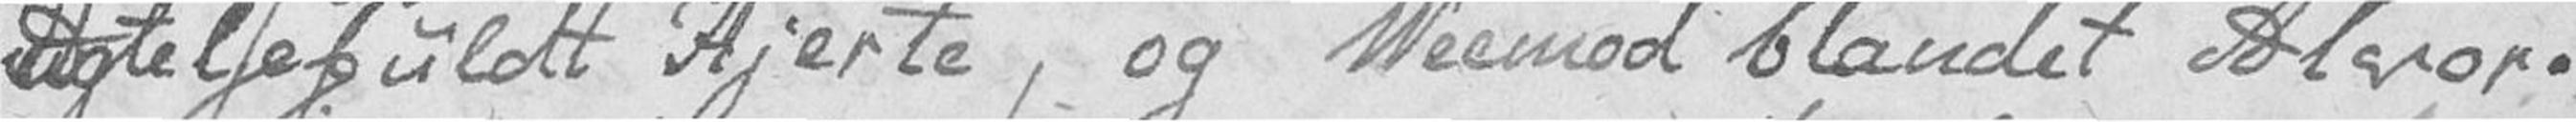Agtelsefuldt Hjerte, og Veemod blandet Alvor.
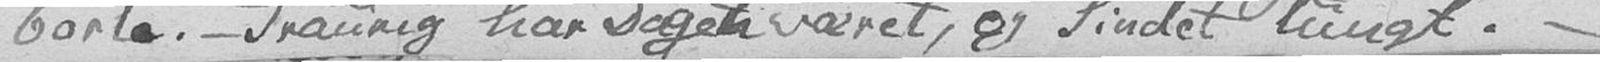borte. – Traurig har Dagen været, og Sindet tungt. –
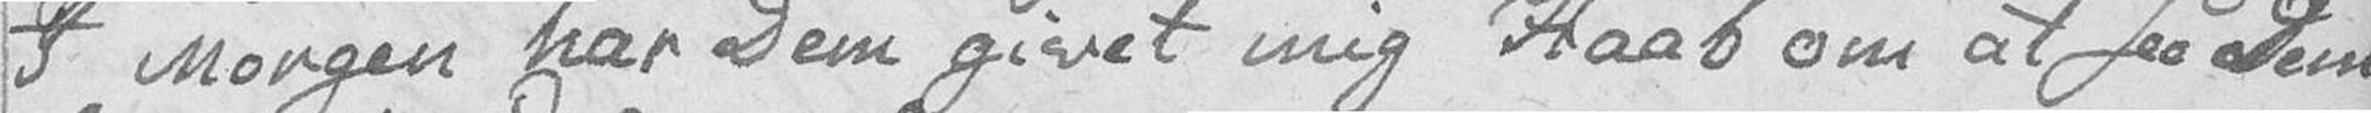I Morgen har Dem givet mig Haab om at see Dem
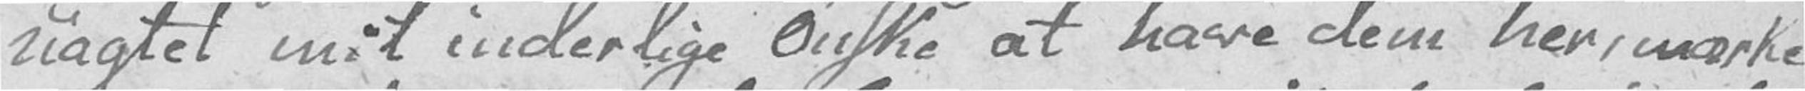uagtet mit inderlige Ønske at have dem her, mærke
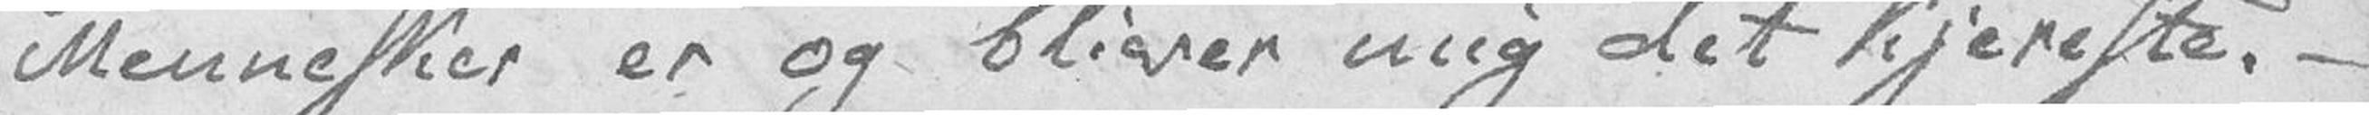Mennesker er og bliver mig det kjereste. –
